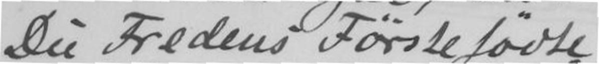Du Fredens Førstefødte,
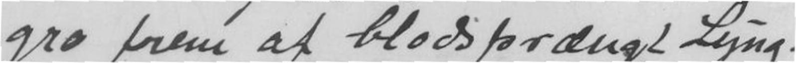gro frem af blodprængt Lyng.
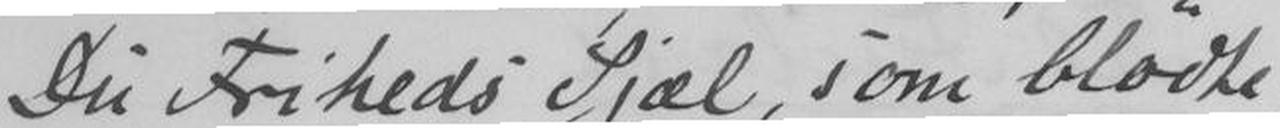Du Friheds Sjæl, som bløde,
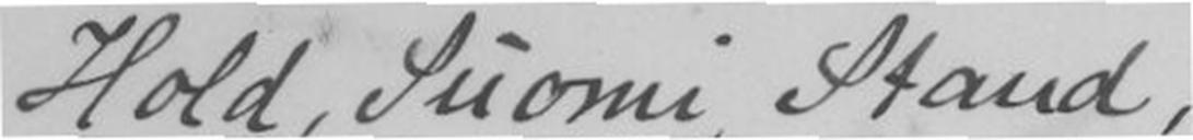Hold, Suomi Stand,
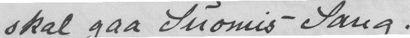skal gaa Suomis Sang.
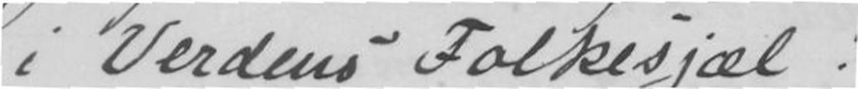i Verdens Folkesjæl!
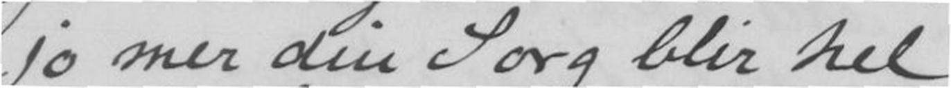jo mer din Sorg blir hel,
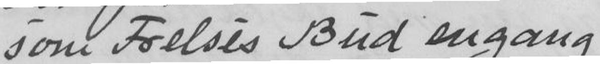som Frelses Bud engang,
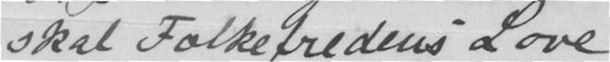skal Folkefredens Love
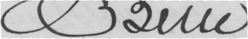JBBull
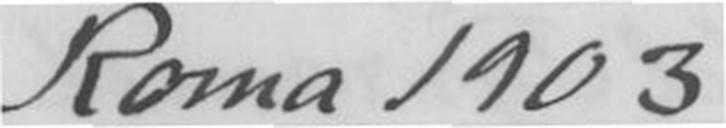Roma 1903
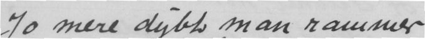Jo mere dybt man rammer,
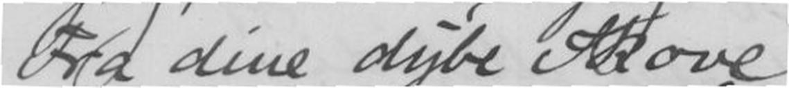Fra dine dybe Skove
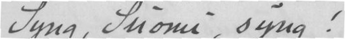Syng, Suomi, syng!
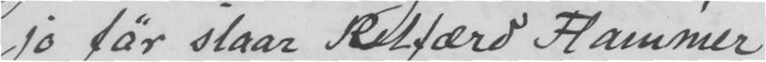jo før slaar Retfærd Flammer
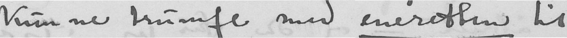kunne trumfe med eneretten til
